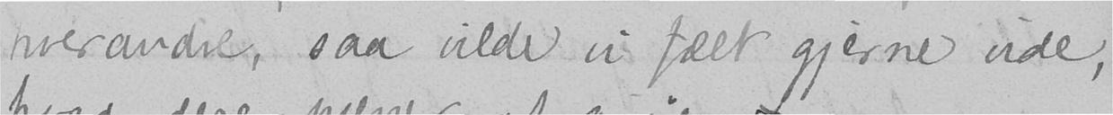hverandre, saa vilde vi fælt gjerne vide,
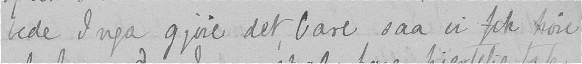bede Inga gjøre det, bare saa vi fik høre
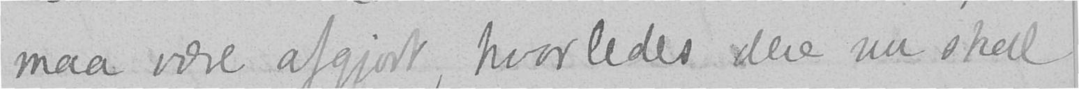maa være afgjort, hvorledes dere nu skal
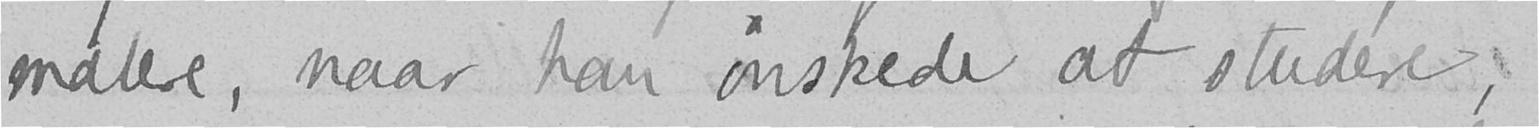malere, naar han ønskede at studere,
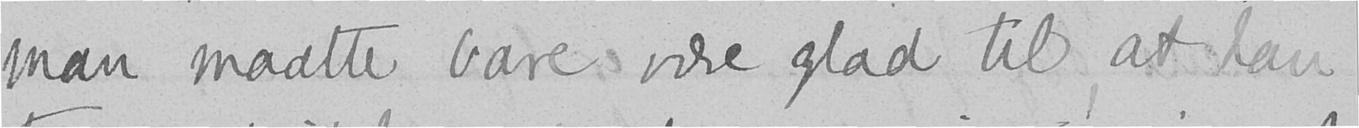man maatte bare være glad til, at han
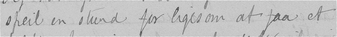speil en stund for ligesom at faa et
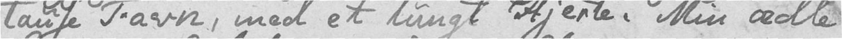tause Favn, med et tungt Hjerte. Min ædle
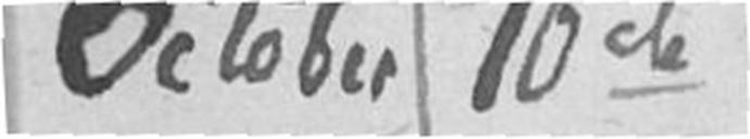October 10de
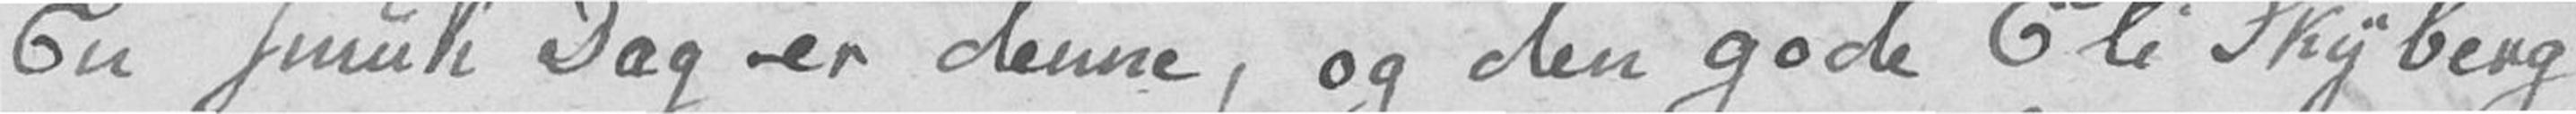En smuk Dag er denne, og den gode Eli Skyberg
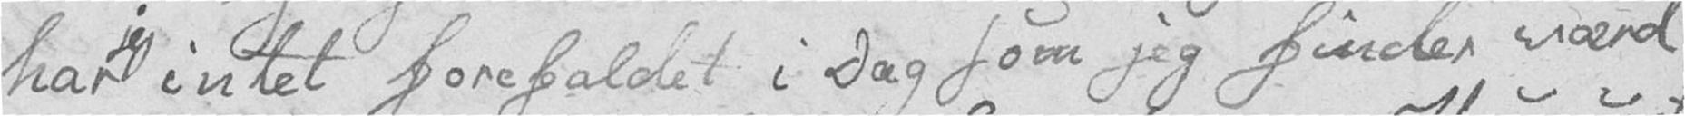har jeg intet forefaldet i Dag som jeg finder værd
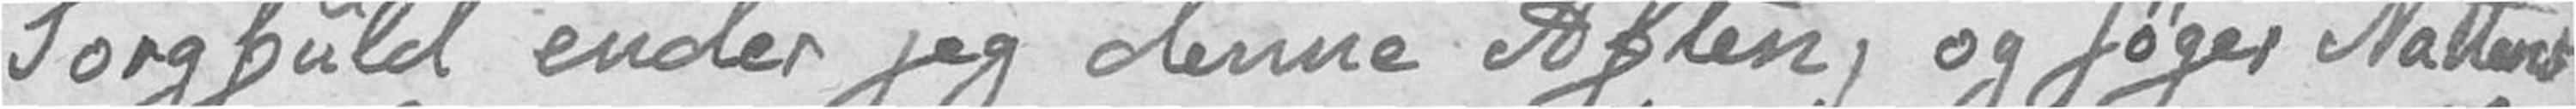Sorgfuld ender jeg denne Aften, og søger Nattens
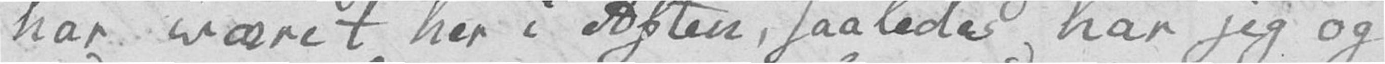har været her i Aften, saaledes har jeg og
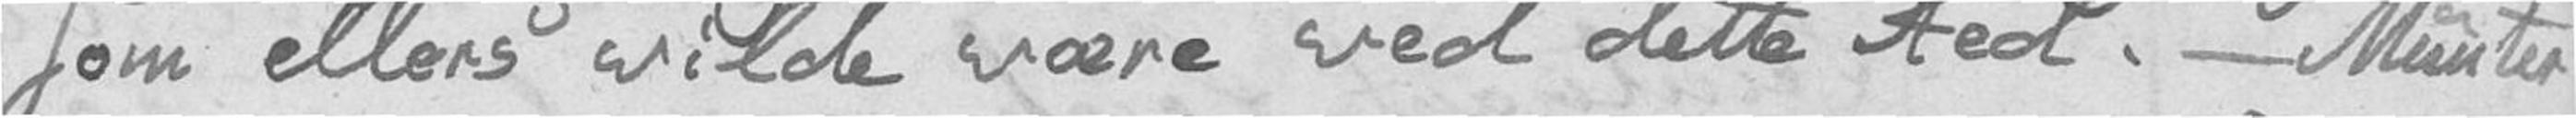som ellers vilde være ved dette Sted. – Munter
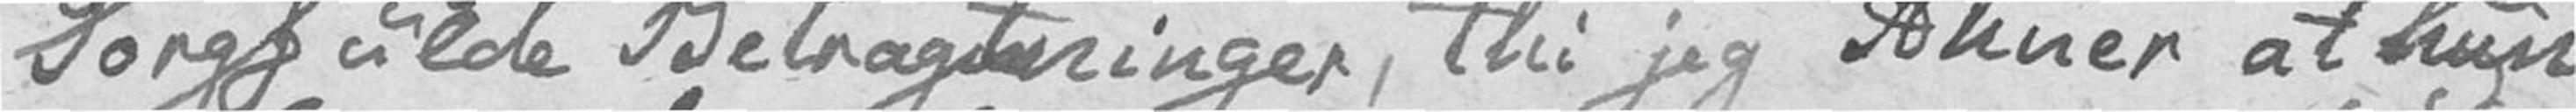Sorgfulde Betragtninger, thi jeg Ahner at hun
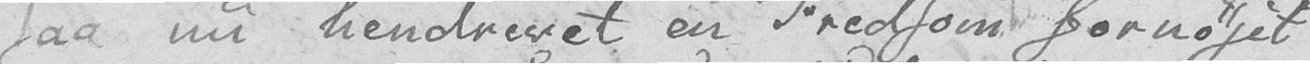saa nu hendrevet en Fredsom fornøjet
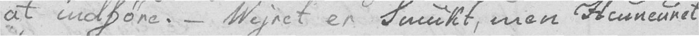at indføre. – Vejret er Smukt, men Humeuret
